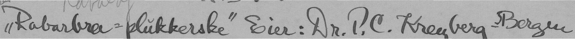"Rabarbra-plukkerske" Eier: Dr. P.C. Kreyberg Bergen
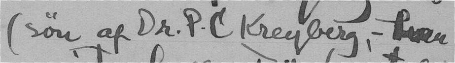(søn af Dr. P.C Kreyberg, - han
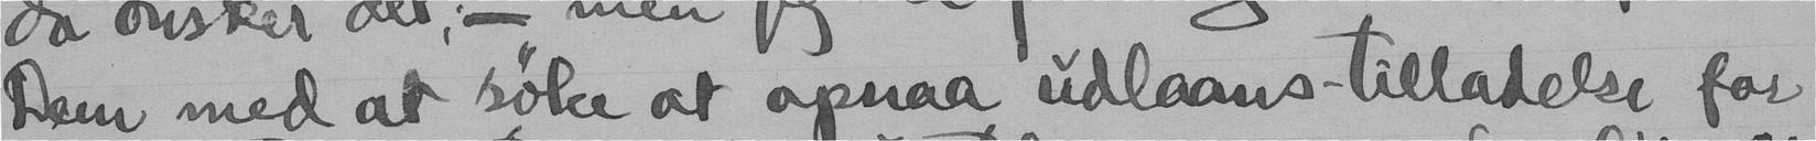dem med at søke at opnaa udlaans-tilladelse for
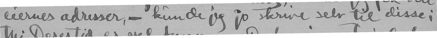eiernes adresser, - kunde jeg jo skrive selv til disse;
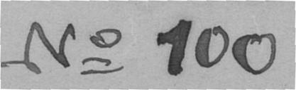No 100
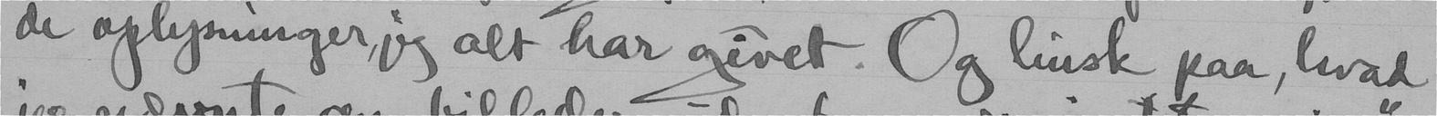de oplysninger, jeg alt har givet. Og husk paa, hvad
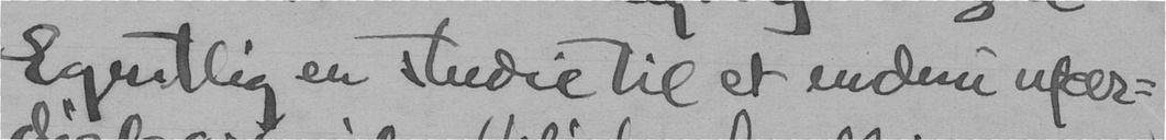Egentlig en studie til et endnu ufær-
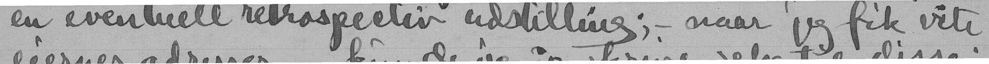en eventuell retrospeetiv udstilling; - naar jeg fik vite
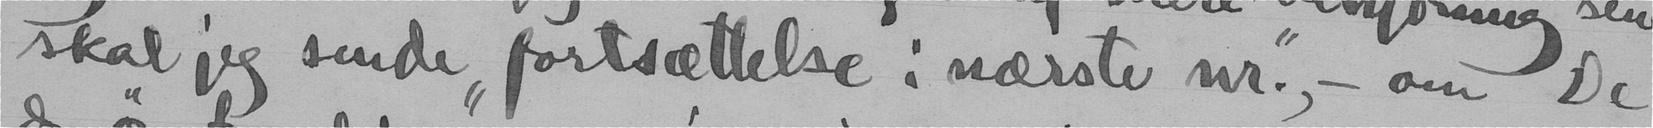skal jeg sende fortsættelse i nærste nr"., - om De
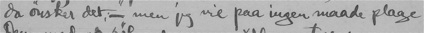da ønsker det, - men jeg vil paa ingen maade plage
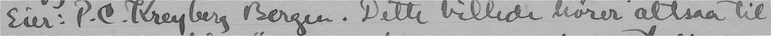Eier: P.C. Kreyberg Bergen. Dette billede hører altsaa til
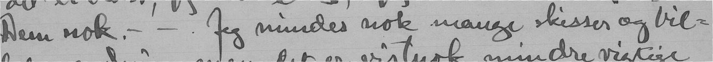Dem nok. - - Jeg mindes nok mange skisser og bil-
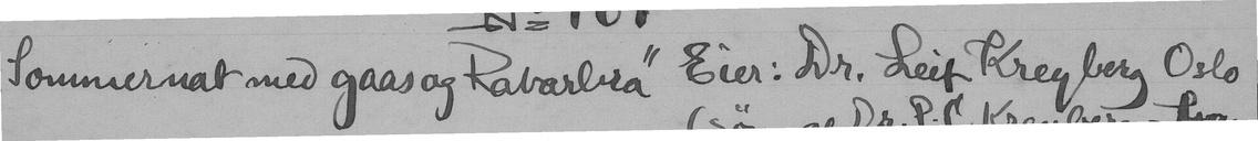"Sommernat med gaas og Rabarbra" Eier. Dr. Leif Kreyberg Oslo
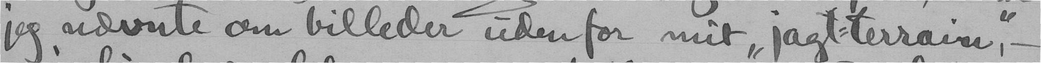jeg nærvnte om billeder udenfor mit "jagt-terrain", -
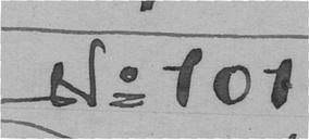No 101
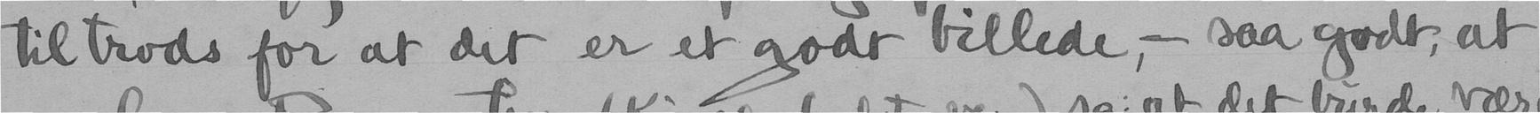tiltrods for at det er et godt billede, - saa godt, at
